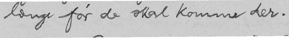længe før de skal komme der.
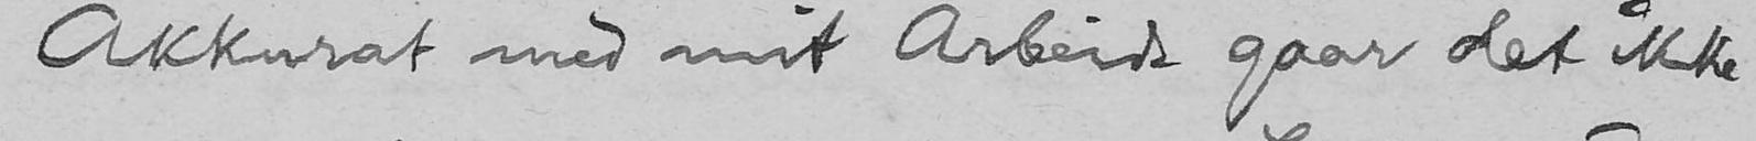Akkurat med mit Arbeide gaar det ikke
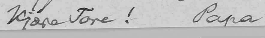Kjære Tore! Papa
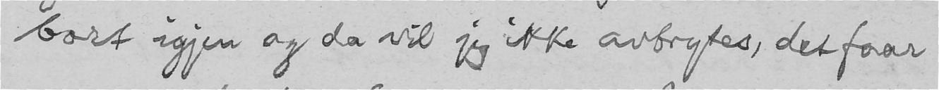bort igjen og da vil jeg ikke avbrytes, det faar
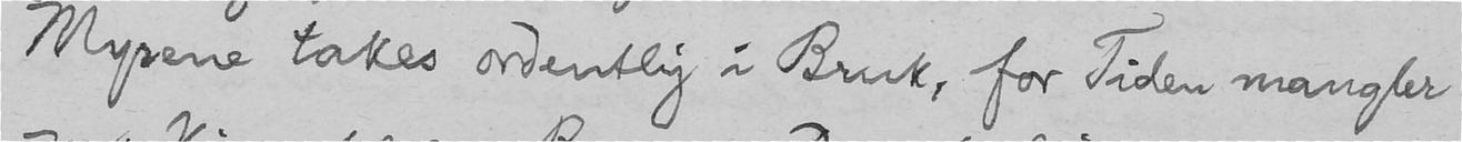Myrene takes ordentlig i Bruk, for Tiden mangler
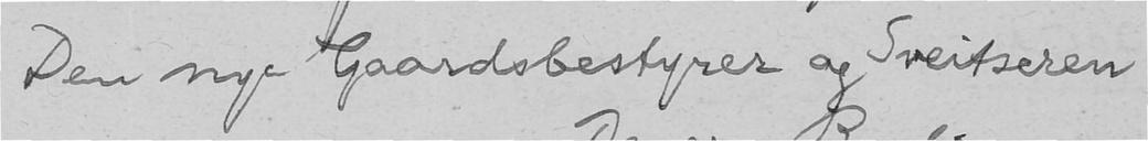Den nye Gaardsbestyrer og Sveitseren
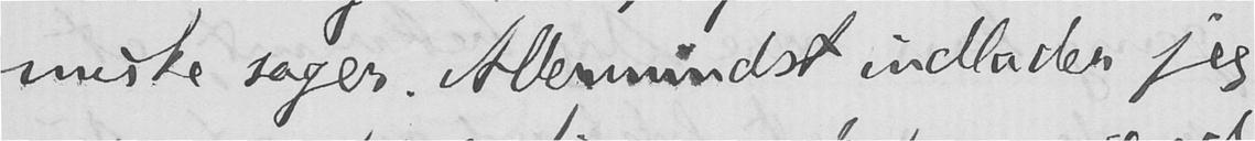miske sager. Allermindst indlader jeg
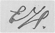EH.
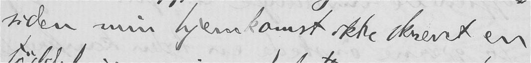siden min hjemkomst ikke skrevet en
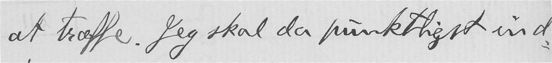at træffe. Jeg skal da punktligst ind-
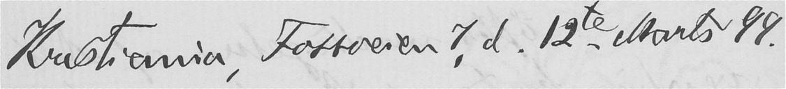Kristiania, Fossveien 7, d. 12te Marts 99.
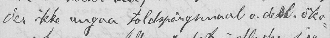der ikke angaa toldspørgsmaal o. desl. øko-
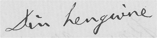Din hengivne
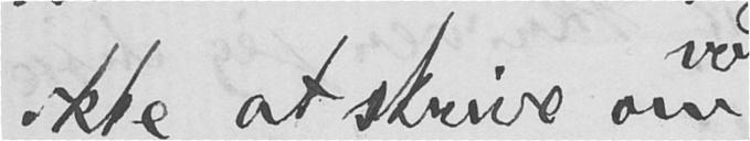ikke at skrive om
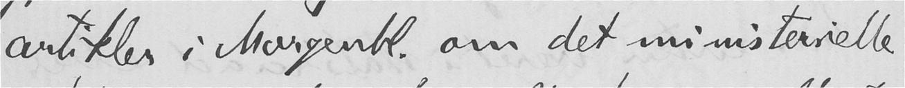artikler i Morgenbl. om det ministerielle
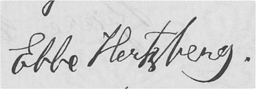Ebbe Hertzberg.
